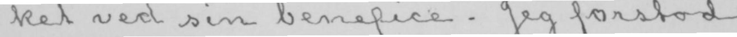ket ved sin benefice. Jeg forstod
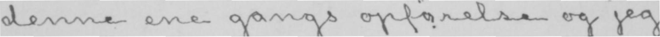denne ene gangs opførelse og jeg
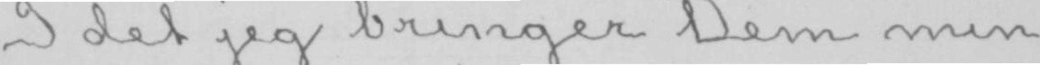I det jeg bringer Dem min
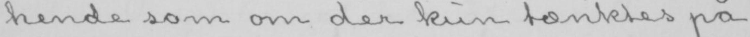hende som om der kun tænktes på
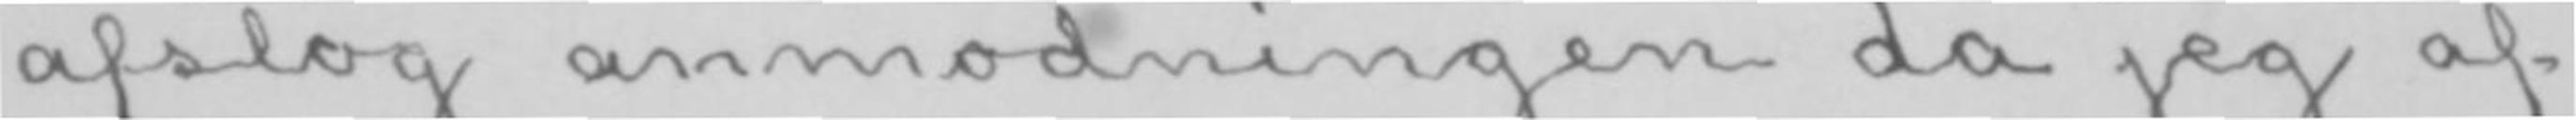afslog anmodningen da jeg af
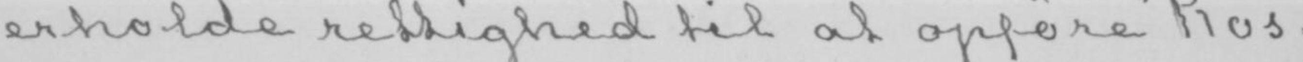erholde rettighed til at opføre «Ros-
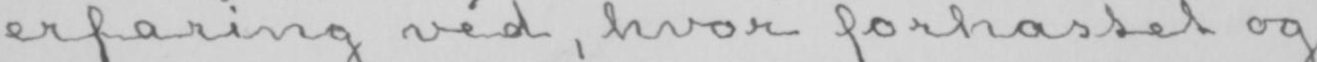erfaring véd, hvor forhastet og
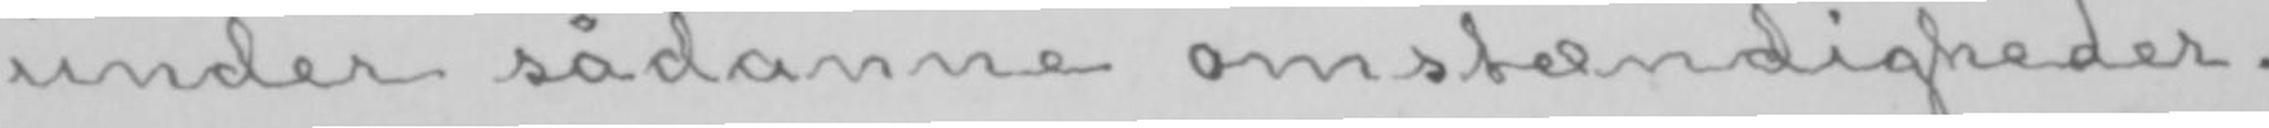under sådanne omstændigheder.
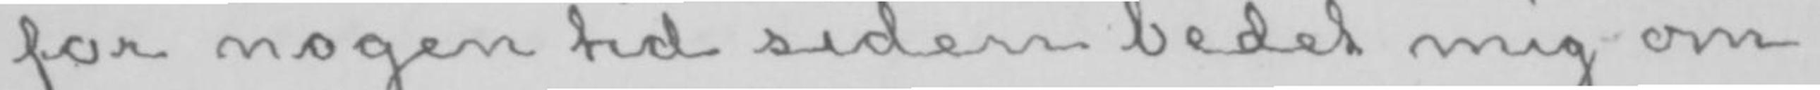for nogen tid siden bedet mig om
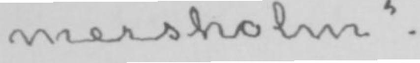mersholm».
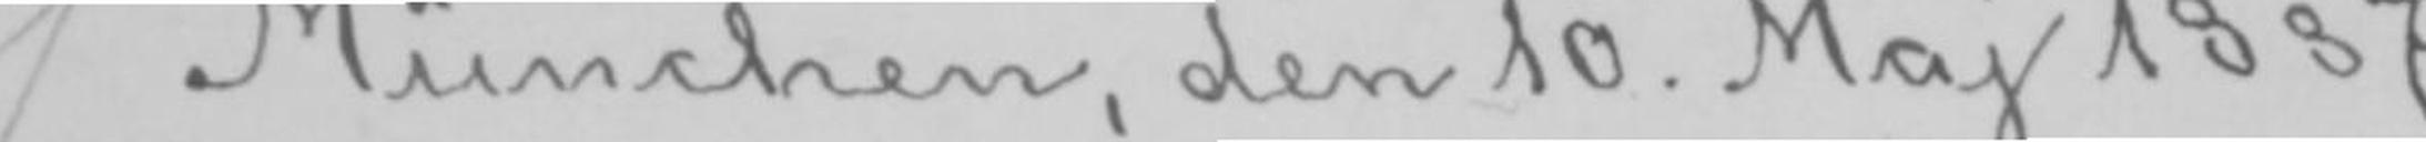München, den 10. Maj 1887.
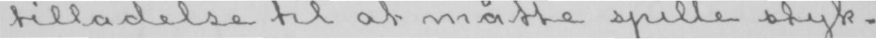tilladelse til at måtte spille styk-
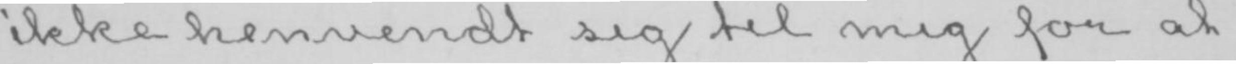ikke henvendt sig til mig for at
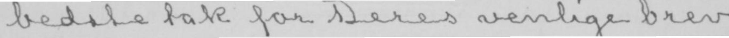bedste tak for Deres venlige brev
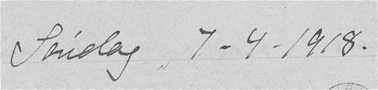Søndag 7-4-1918.
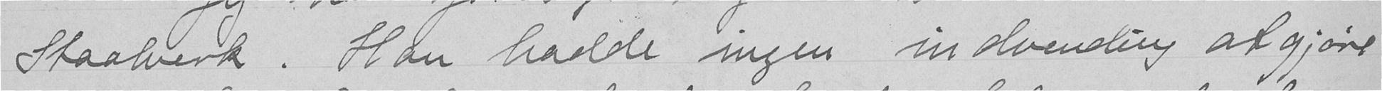Staalverk. Han hadde ingen indvending at gjøre
